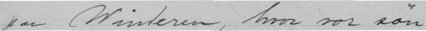paa Winteren, hvor vor søn
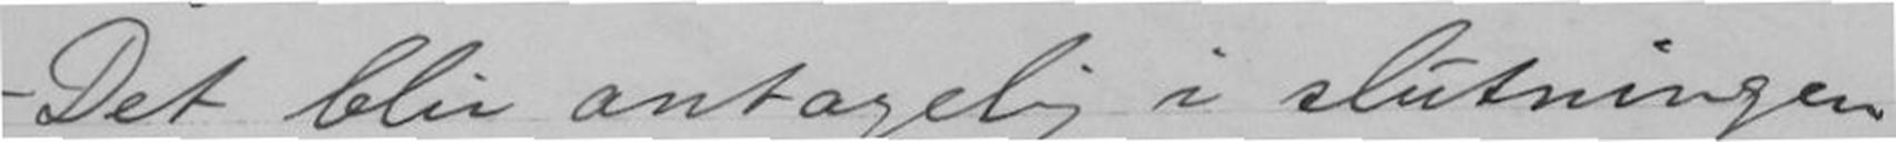- Det blir antagelig i slutningen
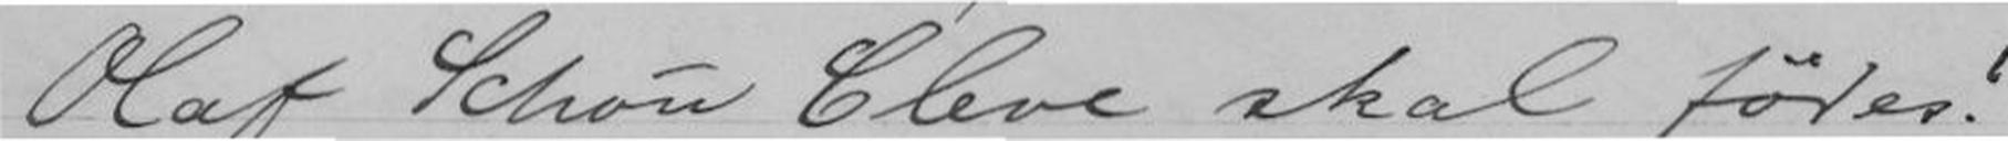Olaf Schou Cleve skal fødes!
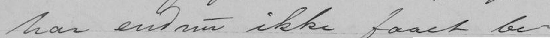har endnu ikke faaet be-
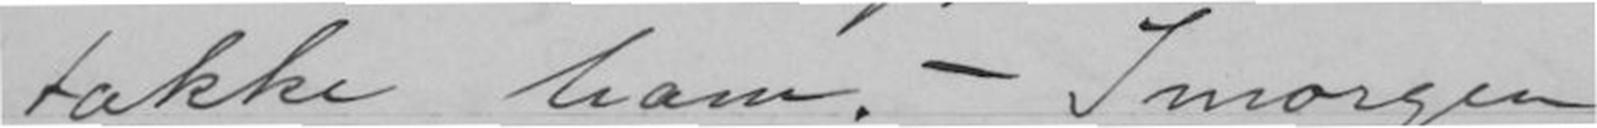takke ham. - Imorgen
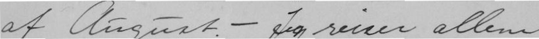af August. - Jeg reiser allene
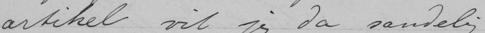artikel vil jeg da sandelig
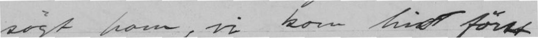søgt ham, vi kom hid først
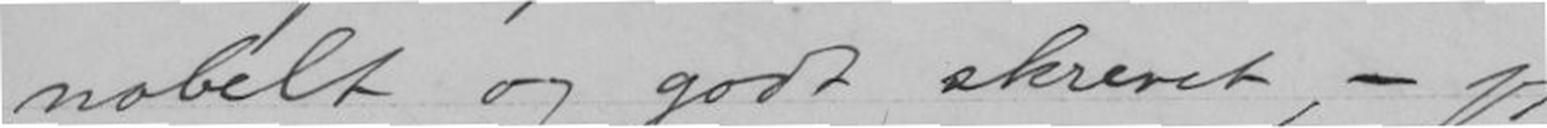nobelt og godt skrevet, - jeg
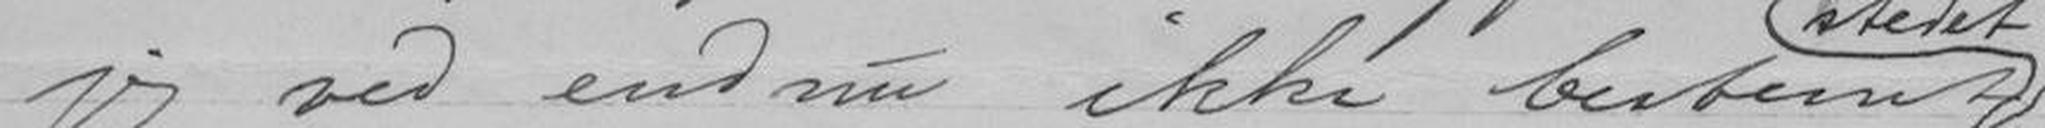jeg ved endnu ikke bestemt
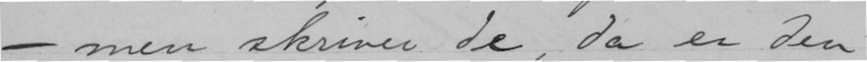- men skriver de, da er den
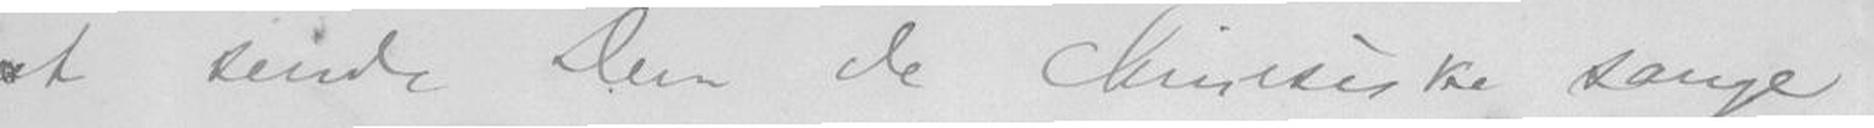at sende Dem de Chinesiske sange,
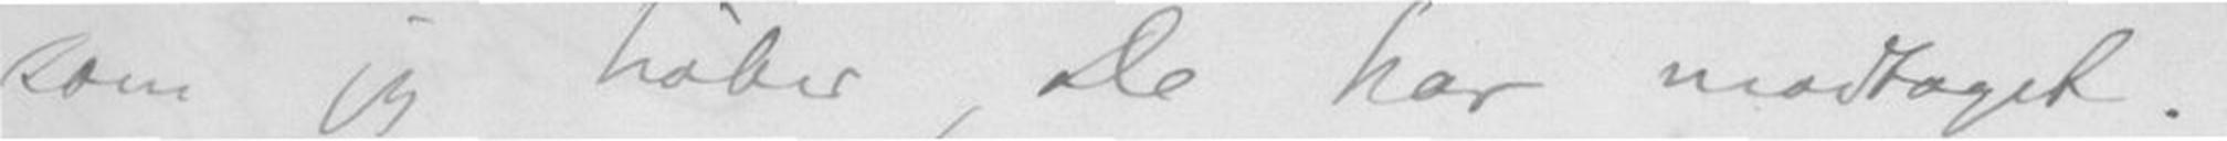som jeg håber De har modtaget.
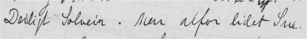Deiligt Solveir. Men alfor lidet Sne.
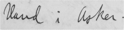Vand i Asker.
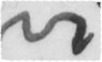vi
